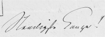Naadigste Konge!
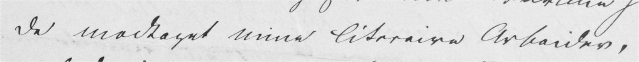de modtaget mine literaire Arbeider,
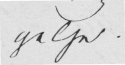gelse.
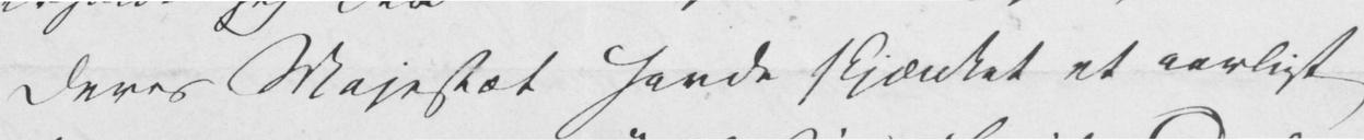Deres Majestæt havde skjænket et aarligt
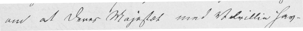om at Deres Majestæt med Velvillie hav-
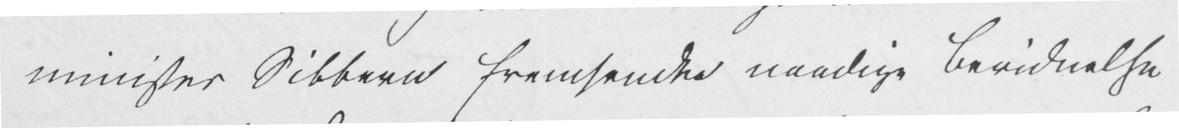minister Sibbern fremsendte naadige Bevidnelse
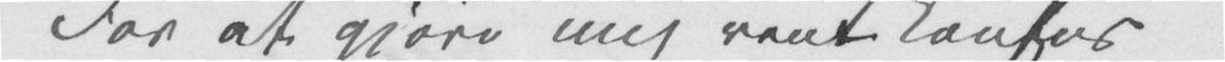For at gjøre mig rent konfus
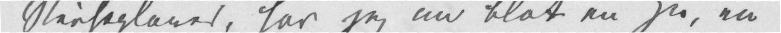Reiseplaner, har jeg nu blot en Hu, en
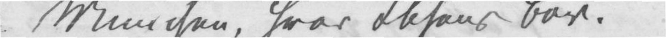München, hvor Ibsens bor.
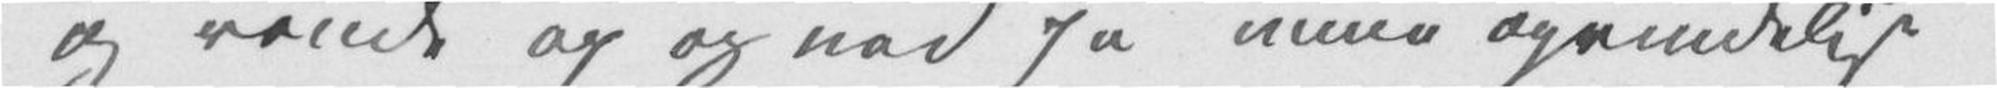og vende op og ned på mine oprindelige
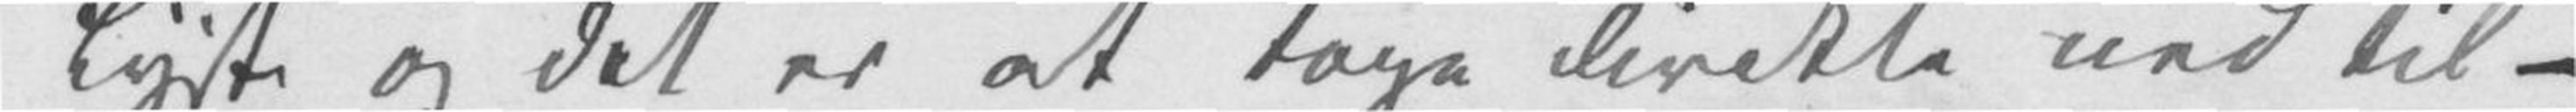Lyst og det er at tage direkte ned til –
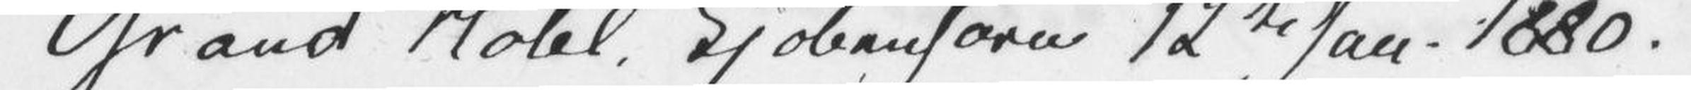Grand Hotel. Kjøbenhavn 12te Jan. 1880.
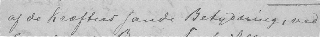af de Kræfters sande Betydning, ved
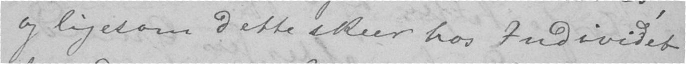og ligesom dette skeer hos Individet
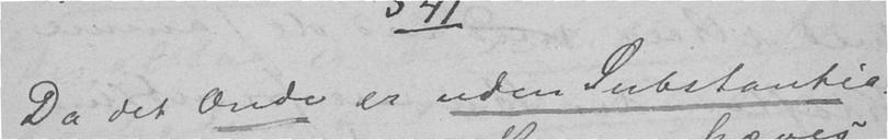Da det Onde er uden Substantia-
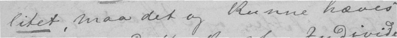litet, maa det og kunne hæves,
